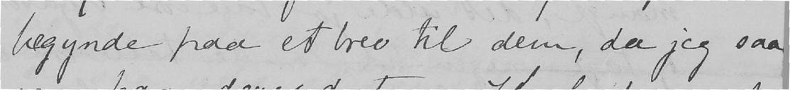begynde paa et brev til dem, da jeg saa
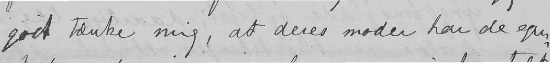godt tænke mig, at deres moder har de egen-
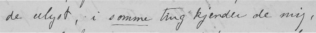de ulyst, i somme ting kjender de mig,
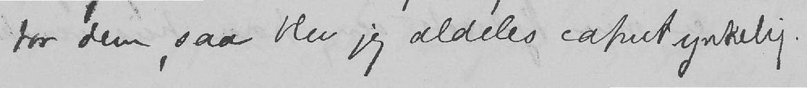for dem, saa blev jeg aldeles caput ynkelig.
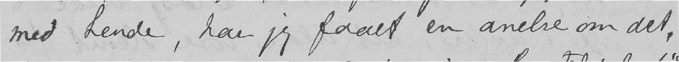med hende, har jeg faaet en anelse om det,
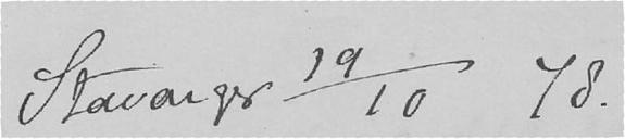Stavanger 19/10 78.
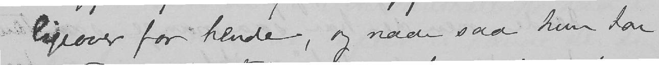ligeover for hende, og naar saa hun har
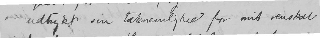udtrykt sin taknemlighed for mit venskab
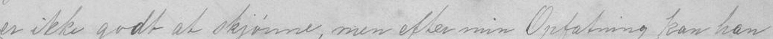er ikke godt at skjønne, men efter min Opfatning kan han
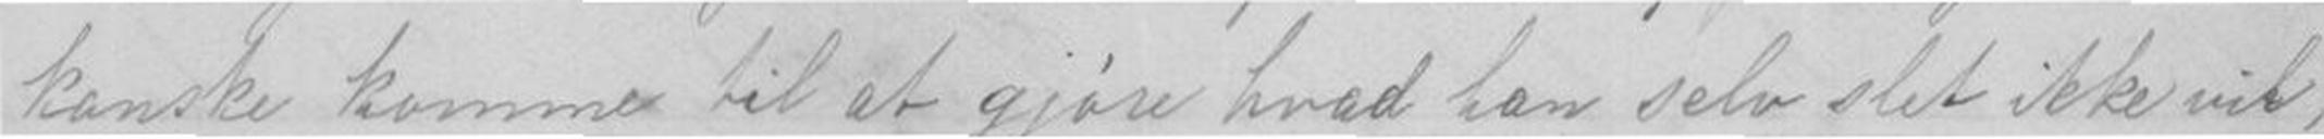kanske komme til at gjøre hvad han selv slet ikke vil,
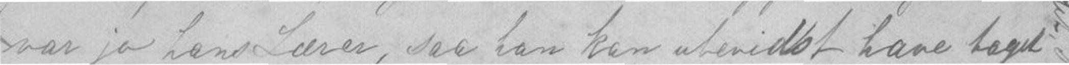var jo hans lærer, saa han kan Ubevidst have taged
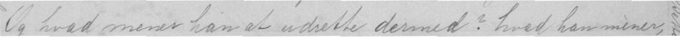Og hvad mener ham at udrette dermed? hvad han mener,
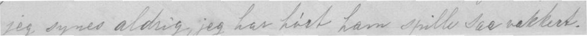jeg synes aldrig jeg har hørt ham spille saa vakkert.
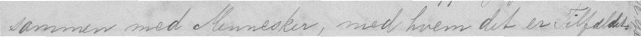sammen med Mennesker, med hvem det en tilfalder.
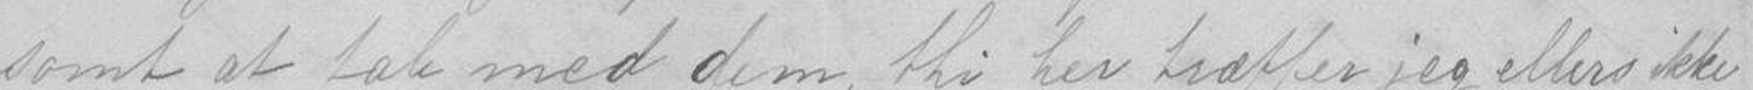somt at tale med dem, thi her træffer jeg ellers ikke
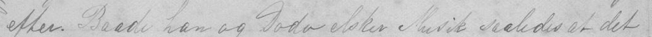efter. Baade han og Dodo elsker Musik saaledes at det
